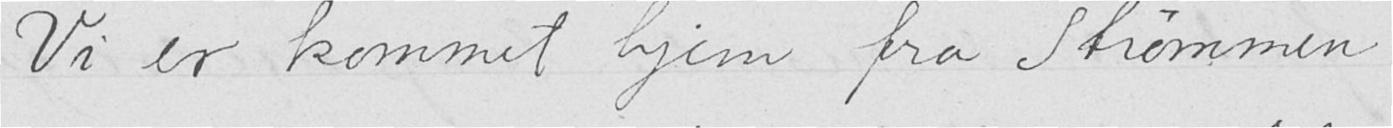— Vi er kommet hjem fra Strømmen
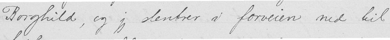Borghild, og jeg slentrer i forveien ned til
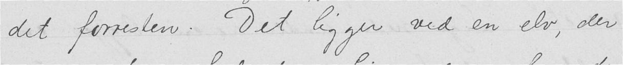det forresten. Det ligger ved en elv, der
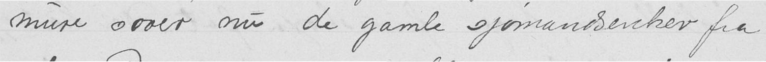mure sover nu de gamle sjømandsenker fra
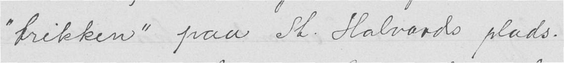"trikken" paa St. Halvards plads.
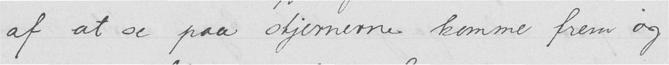af at se paa stjernerne komme frem og
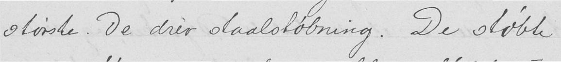største. De drev staalstøbning. De støbte
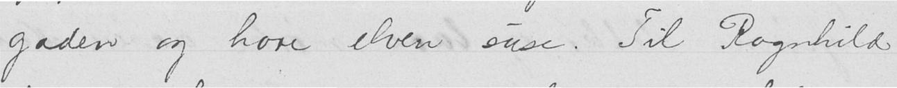gaden og høre elven suse. Til Ragnhild
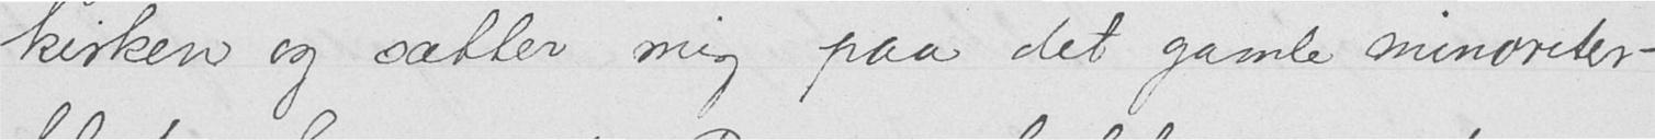kirken og sætter mig paa det gamle minoreter-
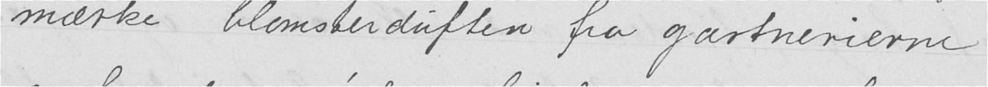mærke blomsterduften fra gartnerierne
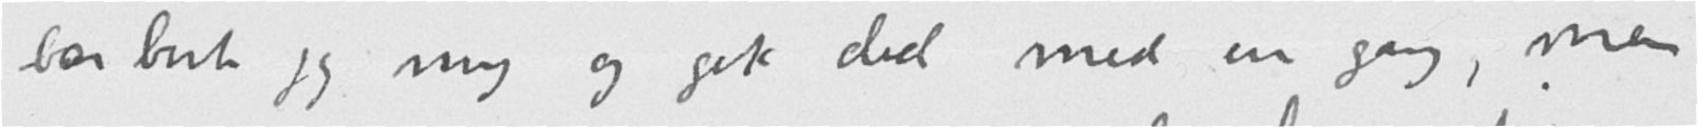barberte jeg mig og gik did med en gang, men
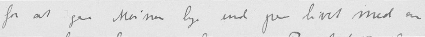for at gaa Meiner lige ind paa livet med en
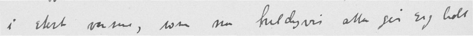i sterk varme, som nu heldigvis atter gir sig lidt
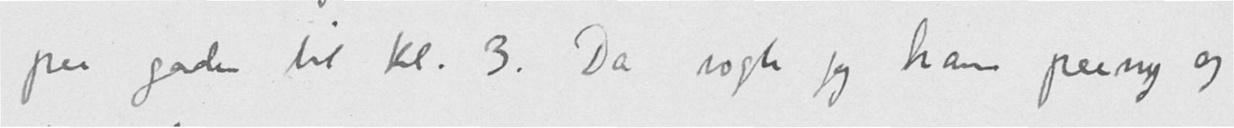paa gaden til kl. 3. De søgte jeg ham paany og
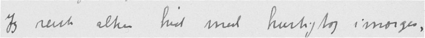Jeg reiste altsaa hid med hurtigtog i morges,
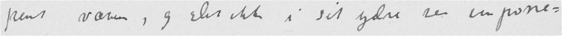pent væsen, og slet ikke i sit ydre saa impone-
